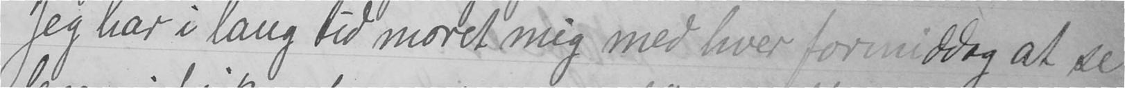Jeg har i lang tid moret mig med hver formiddag at se
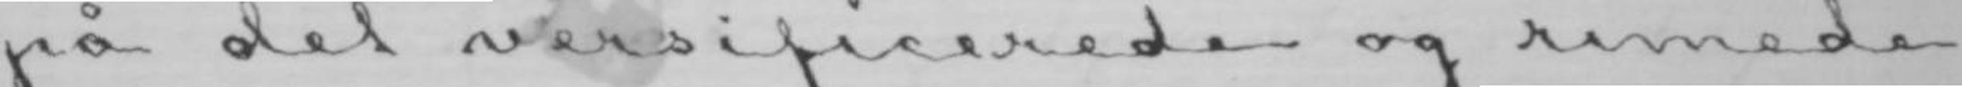på det versificerede og rimede
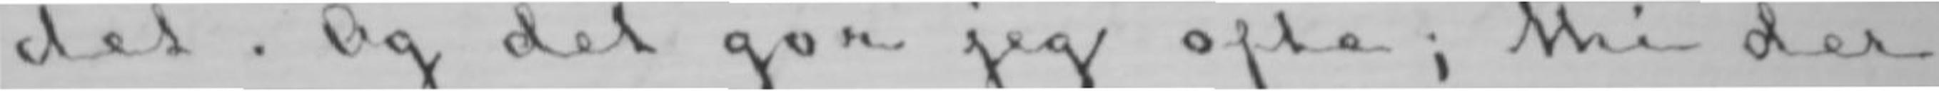det. Og det gør jeg ofte; thi der
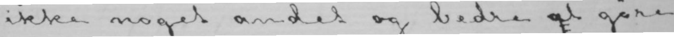ikke noget andet og bedre gat gøre
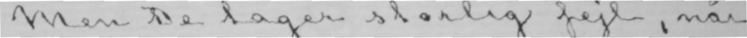Men De tager storlig fejl, når
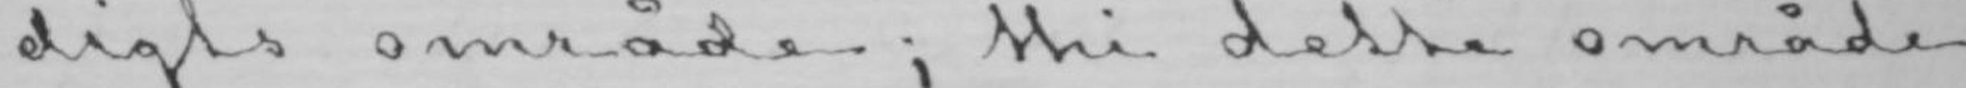digts område; thi dette område
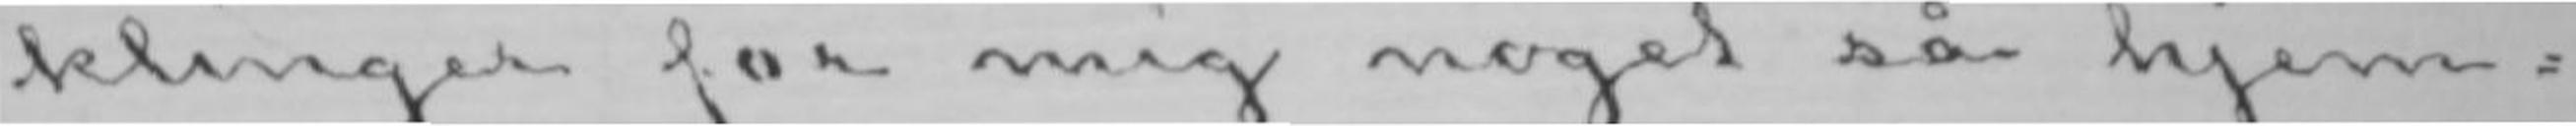klinger for mig noget så hjem-
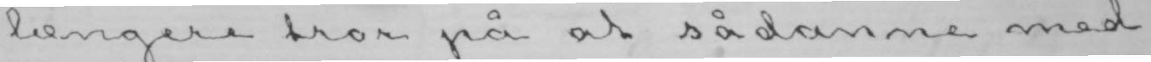længere tror på at sådanne med
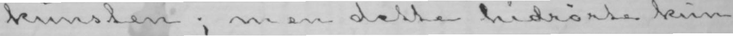kunsten; men dette hidrørte kun
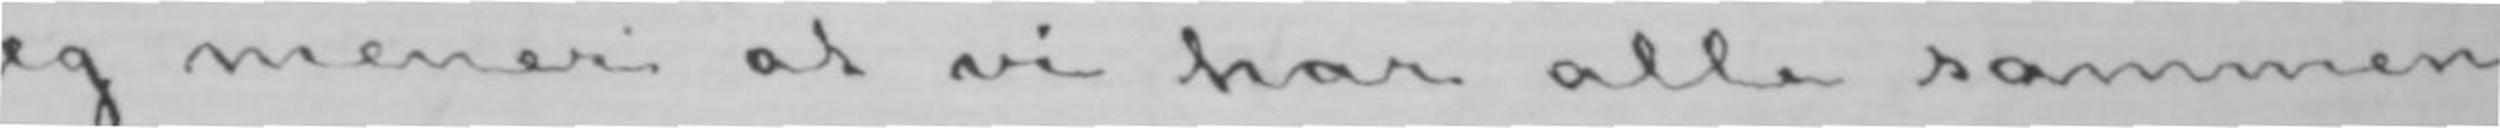Jeg mener at vi har alle sammen
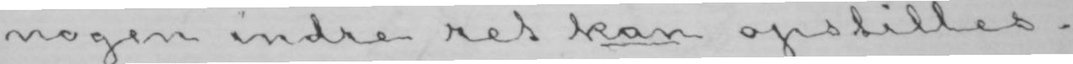nogen indre ret kan opstilles.
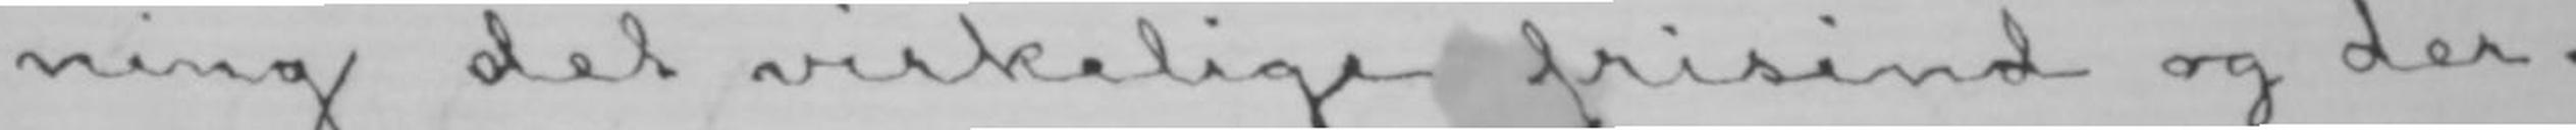ning det virkelige frisind og der-
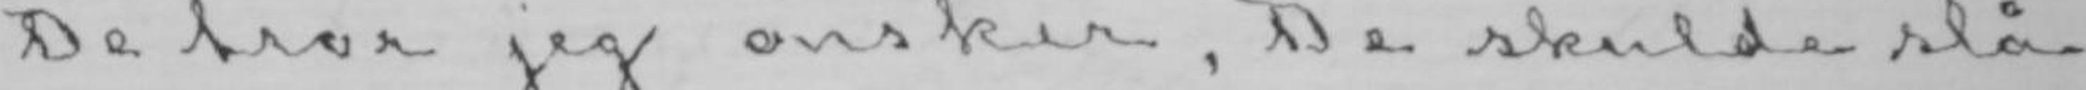De tror jeg ønsker, De skulde slå
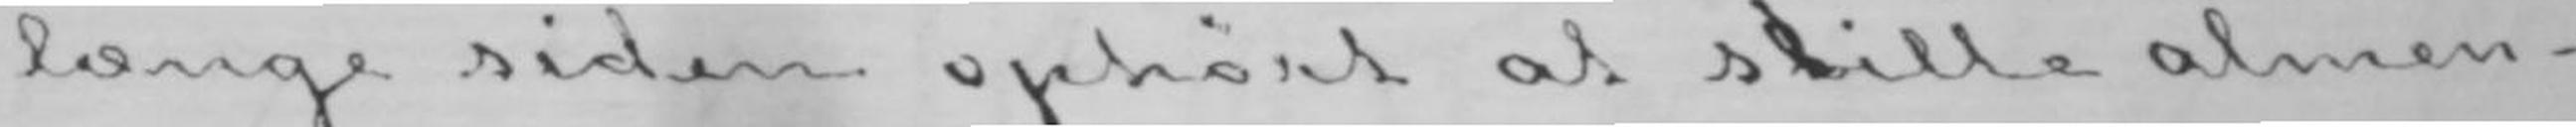længe siden ophørt at stille almen-
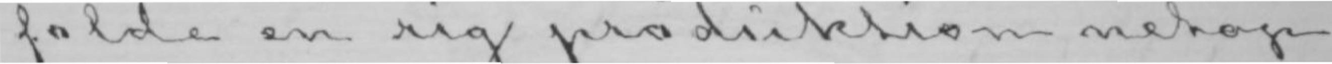folde en rig produktion netop
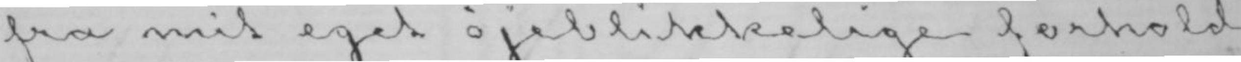fra mit eget øjeblikkelige forhold
